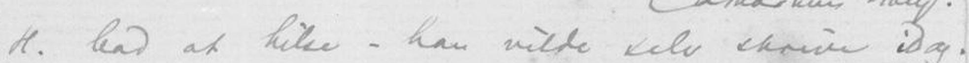H. bad at hilse - han vilde selv skrive idag.
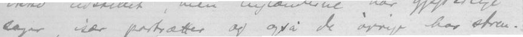sager, især portrætter og også de øvrige har stren-
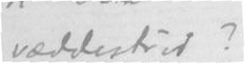væddestrid?
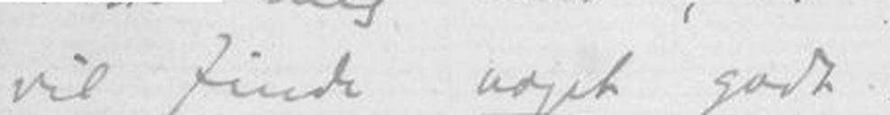vil finde noget godt.
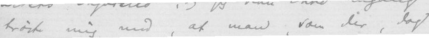trøste mig med, at man, som der, dog
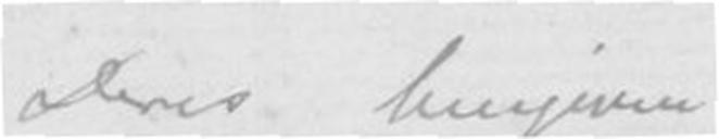Deres hengivne
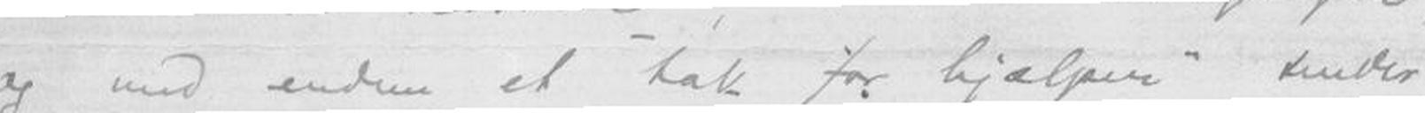og med endnu et "tak for hjælpen" sender
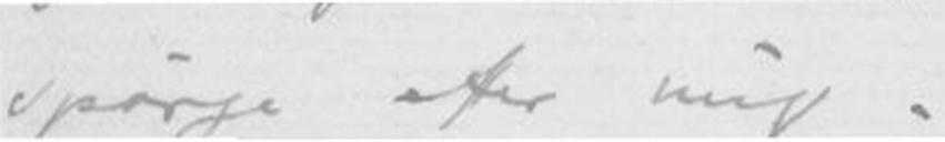spørge efter mig.
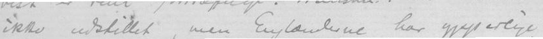ikke udstillet, men Englænderne har ypperlige
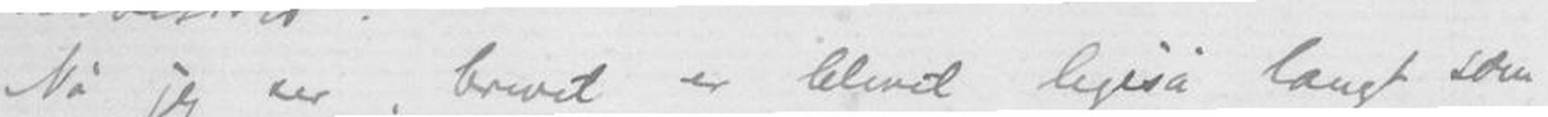Nå jeg ser, brevet er blivet ligeså langt som
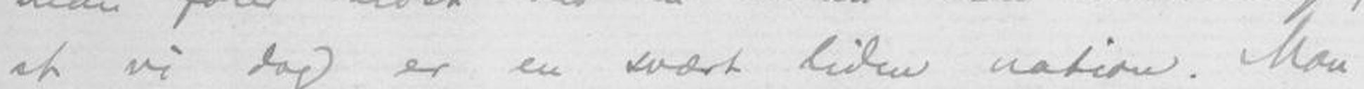at vi dog er en svært liden nation. Mon
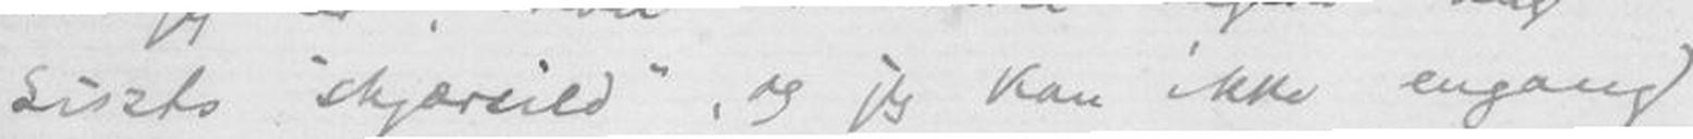sidsts "skjærsild", og jeg kan ikke engang
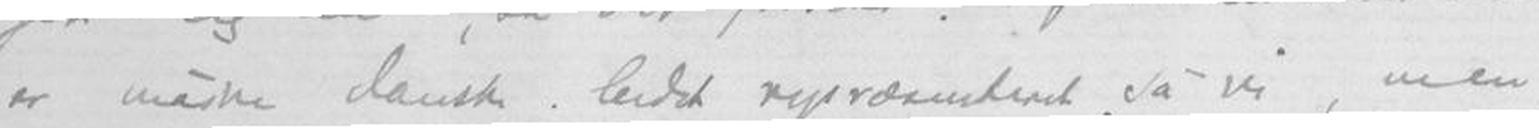er måske danske bedst repræsenteret så vi, men
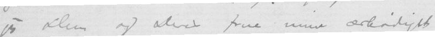jeg Dem og Deres frue mine ærbødigst
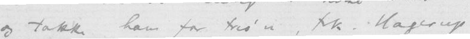og takke ham for trio'n, frk. Hagerup
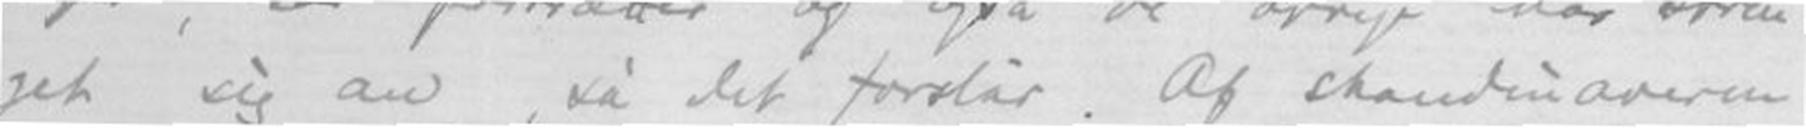get sig an, så det forslår. Af skandinaverne
